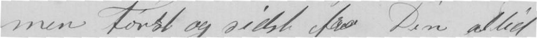men Først og sidst fra Din altid
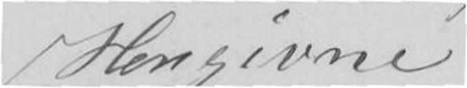Hengivne
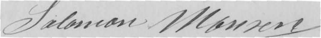Salomon Monsen
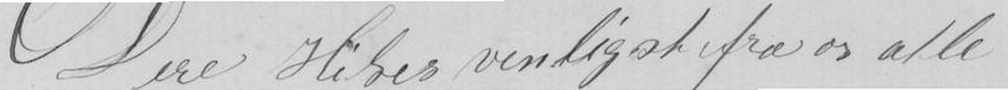Dere Hilses venligst fra os alle
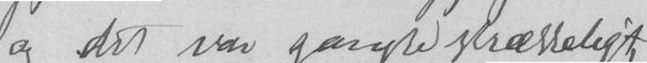og det var ganske skrækkeligt,
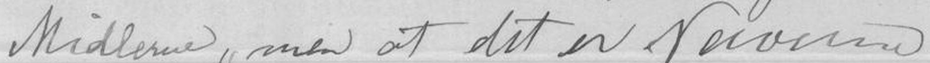Midlerne, men at det er Nærverne
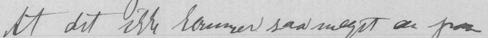At det ikke kommer saa meget i fra
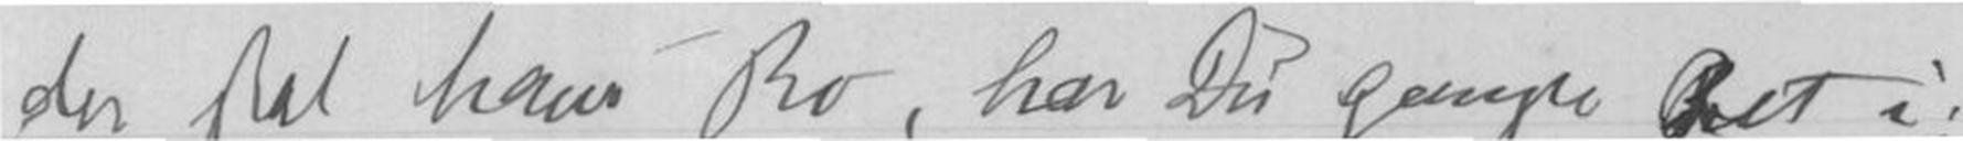der skal have Ro, har Du ganske Ret i;
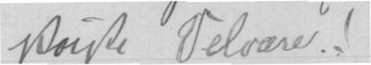største Velvære!
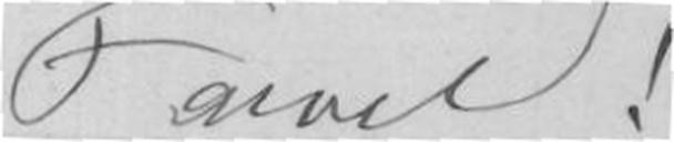Farvel!
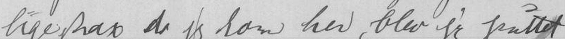ligestrax de jeg kom her, blev jeg puttet
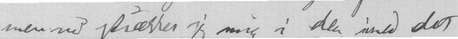men nu strækker jeg mig i den med det
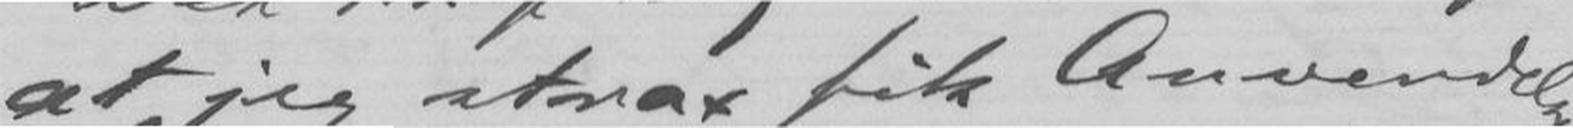at jeg strax fik Anvendelse
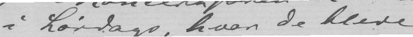i Lørdags, hvor de bleve
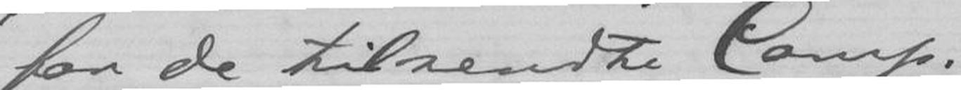for de tilsendte Comp.
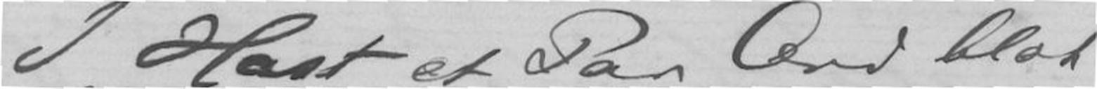I Hast et Par Ord blot
